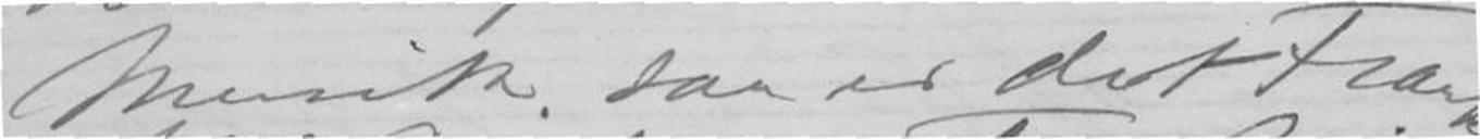Musik, saa er det Frankrig.
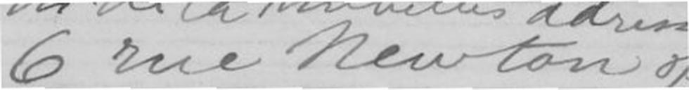6 rue Newton Paris
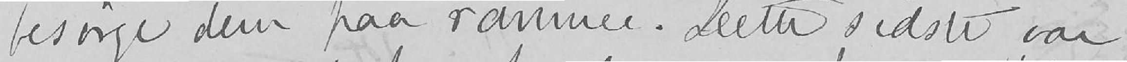besørge dem paa ramme. Dette sidste var
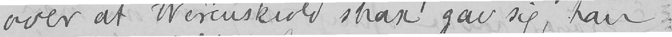over at Werenskiold strax gav sig, han
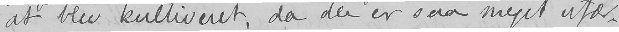at den blev kultiveret, da der er saa meget ufær-
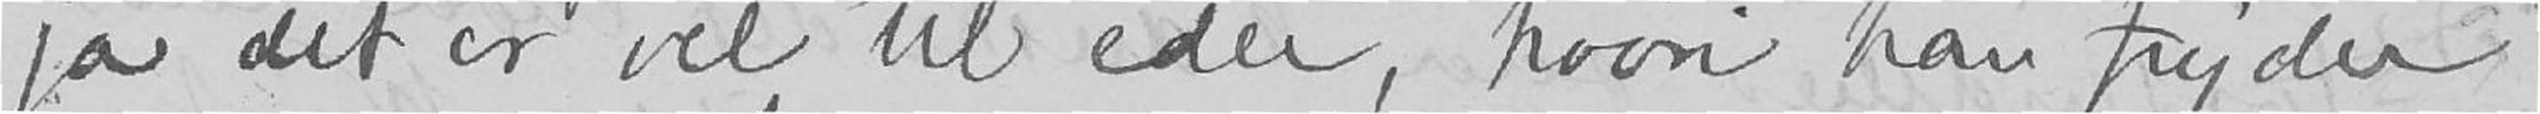ja det er vel til eder, hvori han fryder
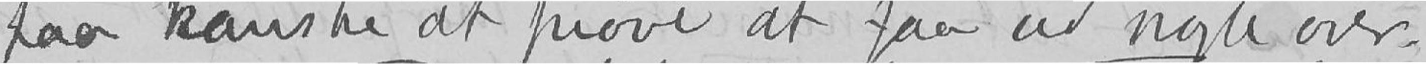paa kanske at prøve at faa ud nogle over-
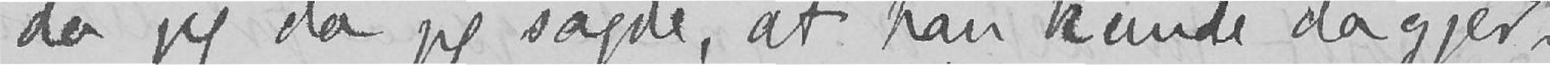da jeg da jeg sagde, at han kunde da gjer-
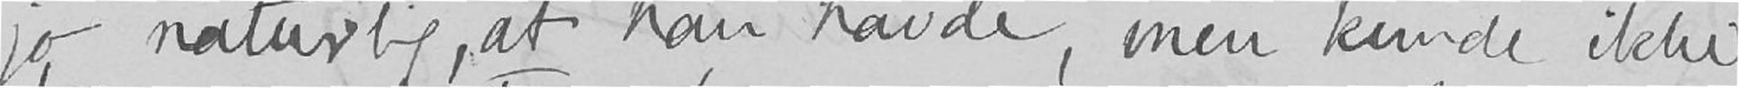jo naturlig, at han havde, men kunde ikke
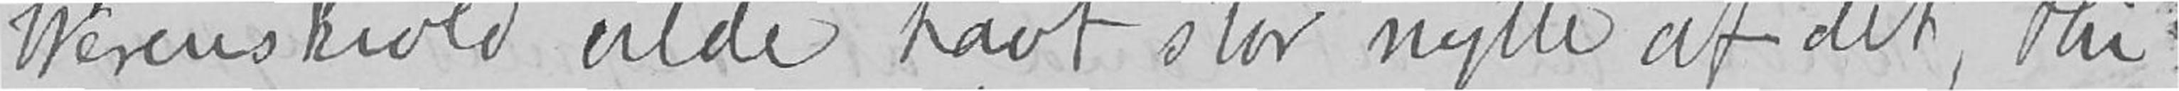Werenskiold vilde havt stor nytte af det, thi
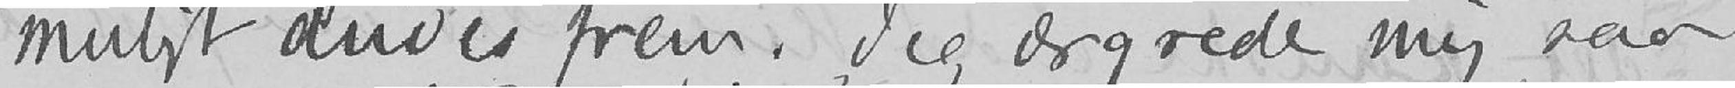muligt drives frem. Jeg ærgrede mig saa,
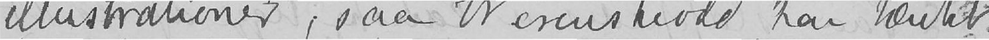illustrationer, saa Werenskiold har tænkt
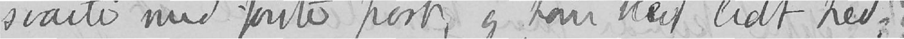svarte med første post, og han blev lidt hed,
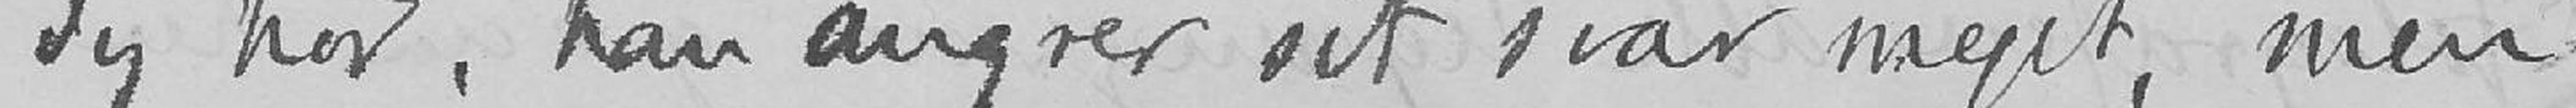Jeg tror, han angrer sit svar meget, men
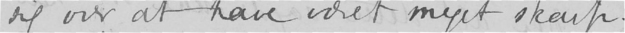sig over at have været meget skarp.
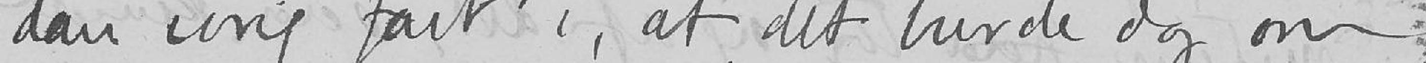dan ivrig fart i, at det burde dog om
